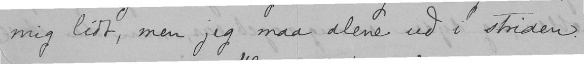mig lidt, men jeg maa alene ud i striden.
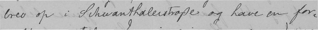brev op i Schwanthalerstraße og have en for-
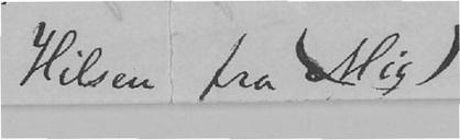Hilsen fra Mig.
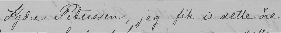Kjære Peterssen, jeg fik i dette øie-
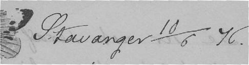Stavanger 10/6 76.
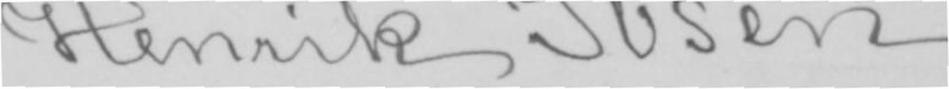Henrik Ibsen.
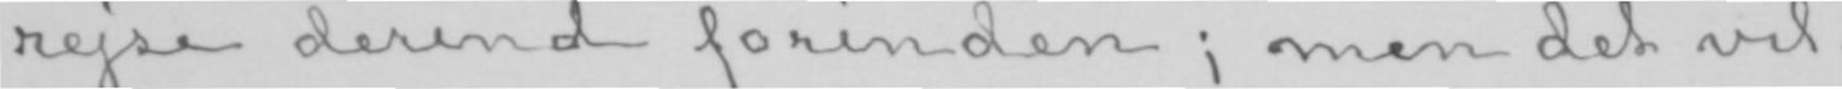rejse derind forinden; men det vil-
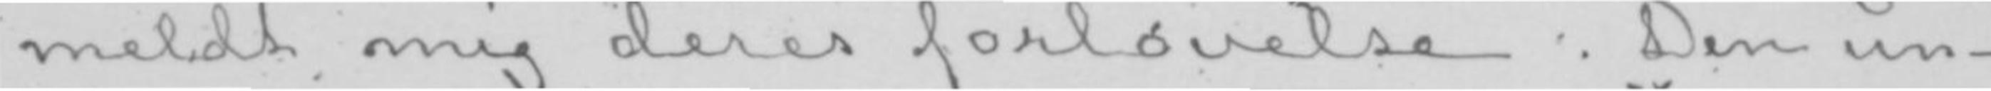meldt mig deres forlovelse. Den un-
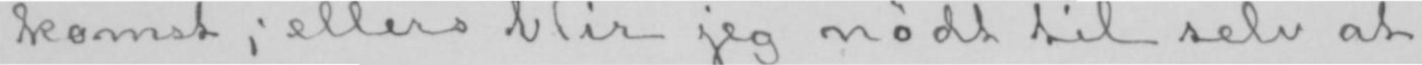komst; ellers blir jeg nødt til selv at
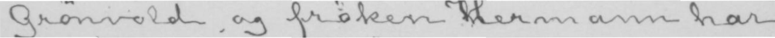Grønvold og frøken Hermann har
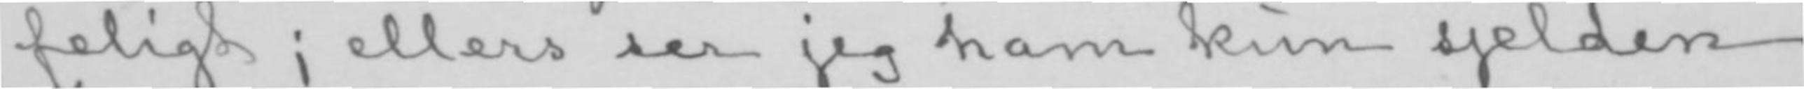feligt; ellers ser jeg ham kun sjelden.
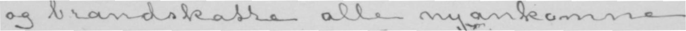og brandskatte alle nyankomne
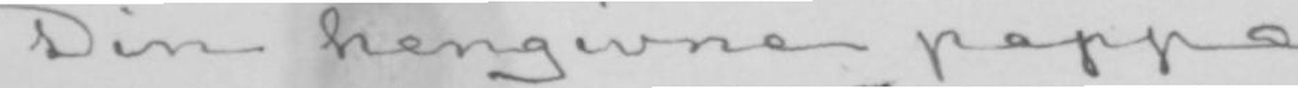Din hengivne pappa
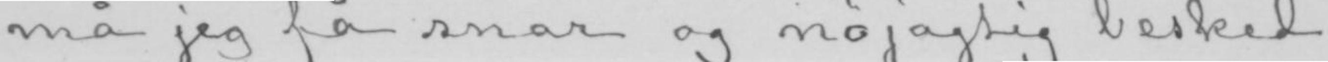må jeg få snar og nøjagtig besked.
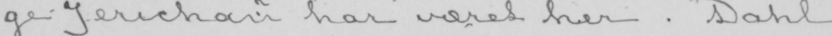ge Jerichau har været her. Dahl
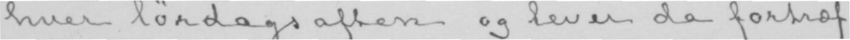hver lørdags aften og lever da fortræf-
